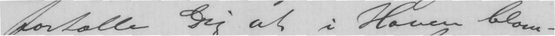fortælle Dig at i Haven blom-
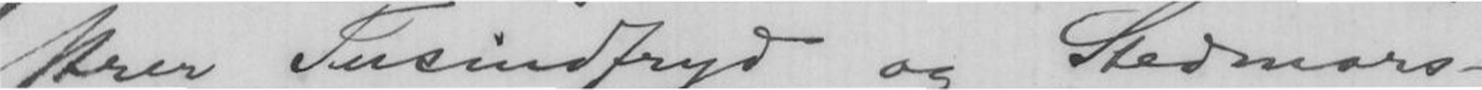strer Tusindfryd og Stedmors-
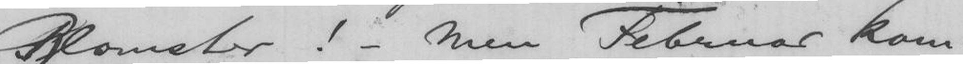Blomster! - Men Februar kom-
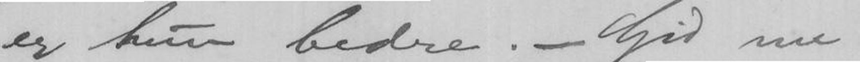er hun bedre. - Gid nu
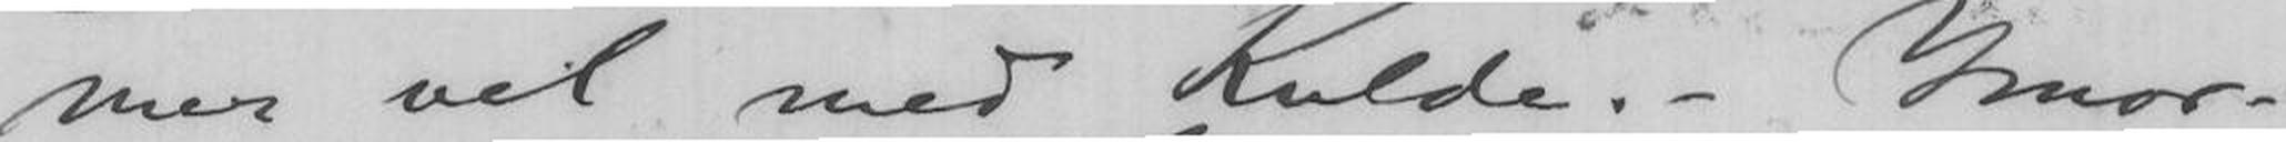mer vel med Kulde. - Imor-
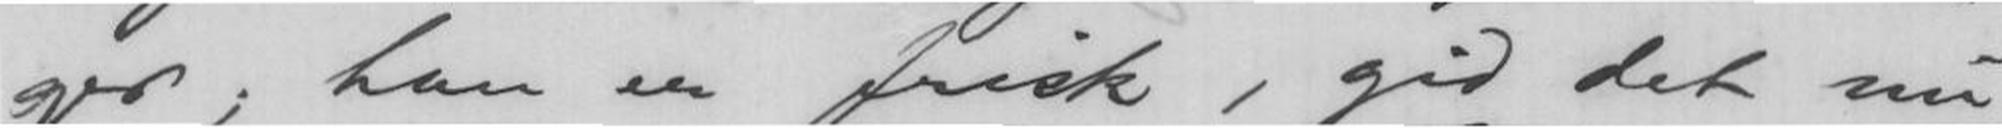ger; han er frisk, gid det nu
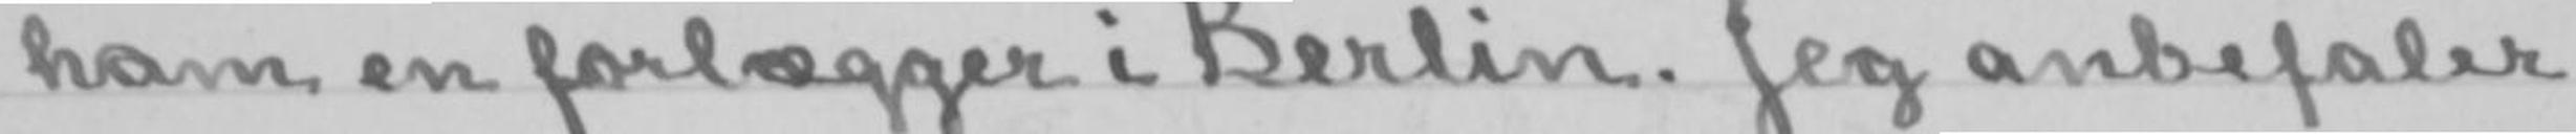ham en forlægger i Berlin. Jeg anbefaler
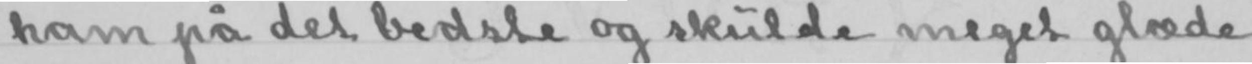ham på det bedste og skulde meget glæde
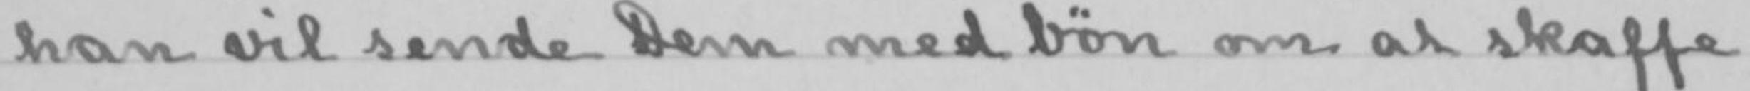han vil sende Dem med bøn om at skaffe
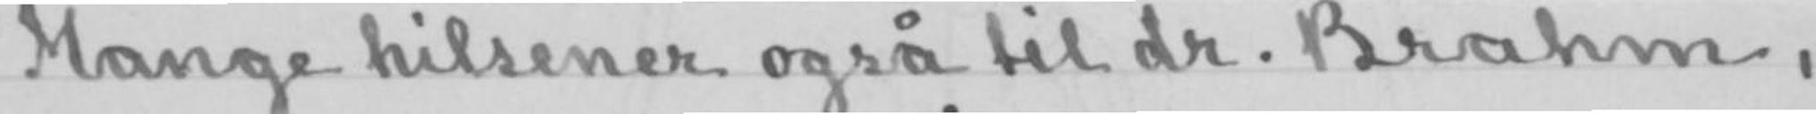Mange hilsener også til dr. Brahm,
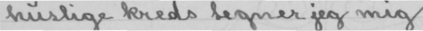huslige kreds tegner jeg mig
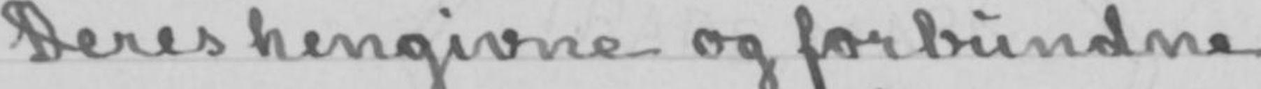Deres hengivne og forbundne
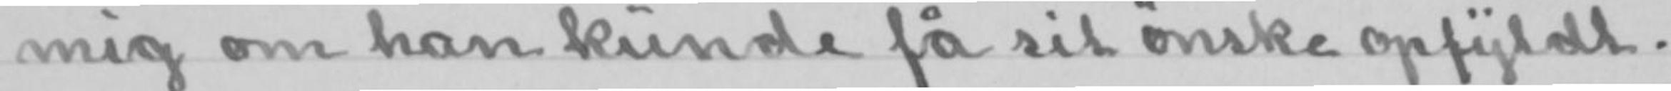mig om han kunde få sit ønske opfyldt.
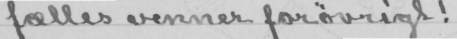fælles venner forøvrigt!
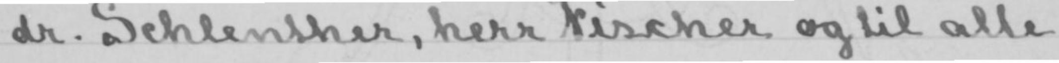dr. Schlenther, herr Fischer og til alle
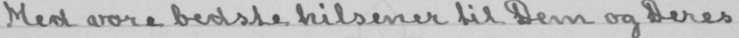Med vore bedste hilsener til Dem og Deres
